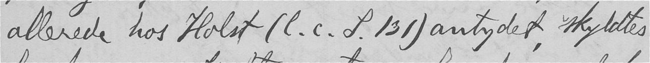allerede hos Holst (l. c. S. 131) antydet, skyldtes
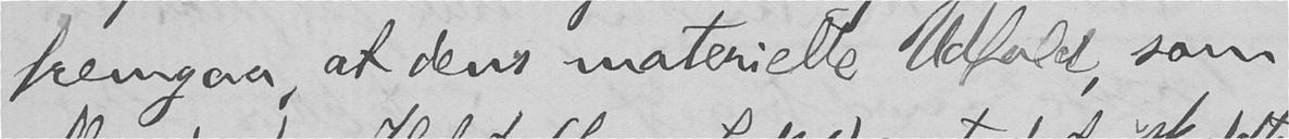fremgaa, at dens materielle Udfald, som
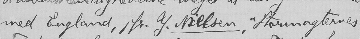med England, jfr. Y. Nielsen, "Stormagternes
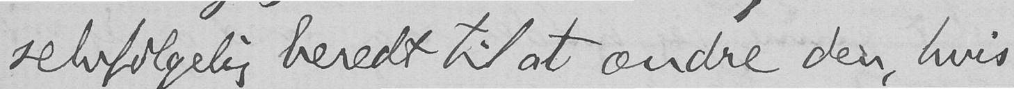selvfølgelig beredt til at ændre den, hvis
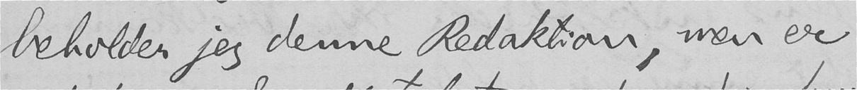beholder jeg denne Redaktion, men er
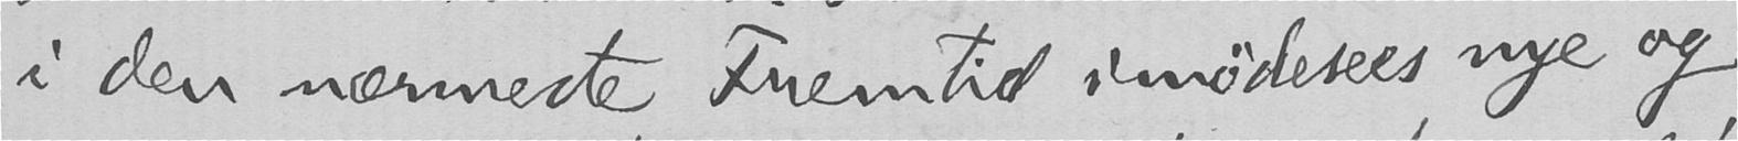i den nærmeste Fremtid imødesees nye og
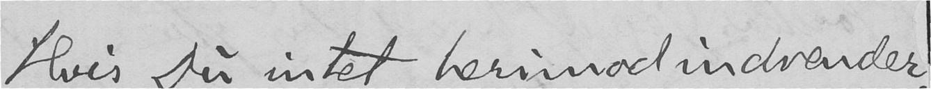Hvis Du intet herimod indvender,
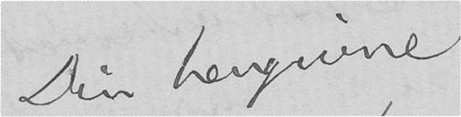Din hengivne
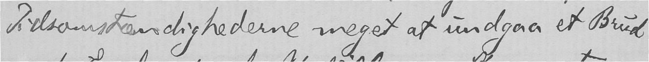Tidsomstændighederne meget at undgaa et Brud
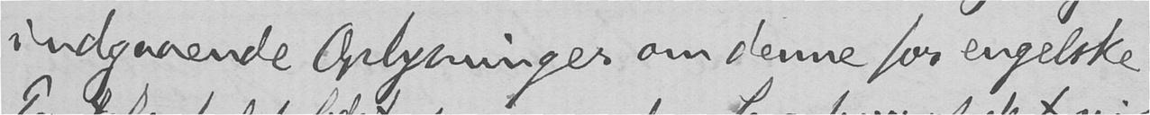indgaaende Oplysninger om denne for engelske
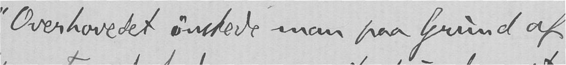"Overhovedet ønskede man paa Grund af
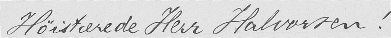Høistærede Herr Halvorsen!
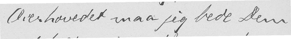Overhovedet maa jeg bede Dem
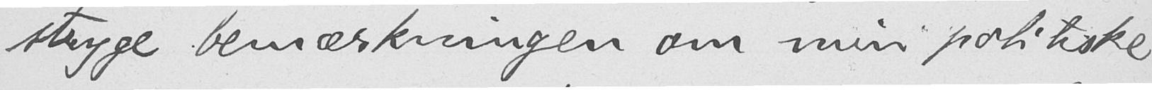stryge bemærkningen om min politiske
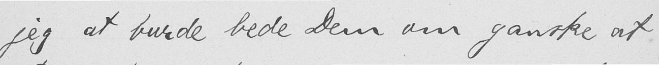jeg at burde bede Dem om ganske at
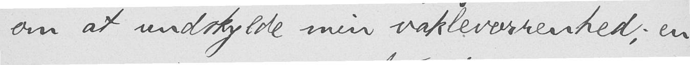om at undskylde min vaklevorrenhed; en
